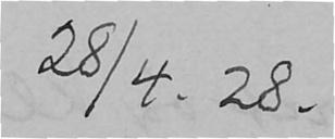28/4. 28.
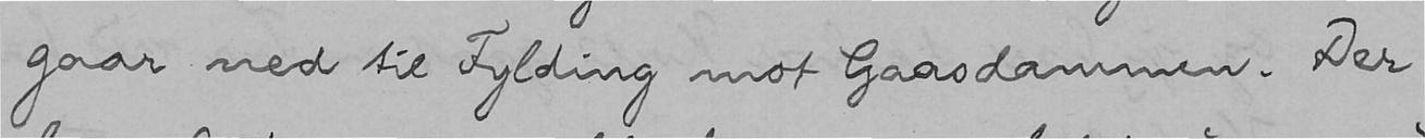gaar med til Fylding mot Gaasdammen. Der
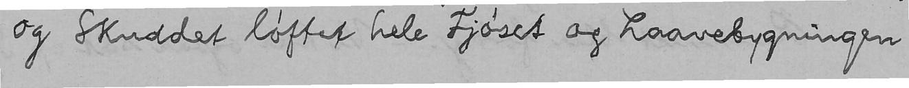og Skuddet løftet hele Fjøset og Laavebygningen
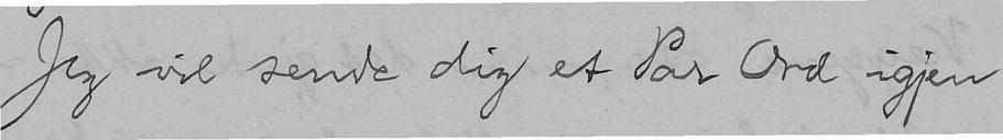Jeg vil sende dig et Par Ord igjen
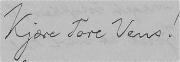Kjære Tore Vens!
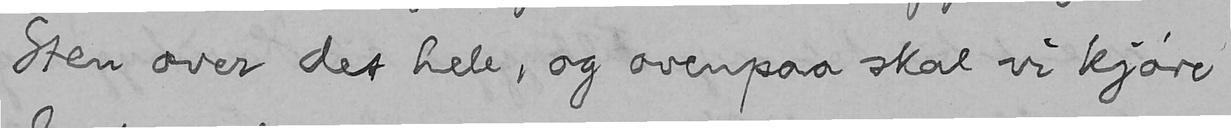Sten over det hele, og ovenpaa skal vi kjøre
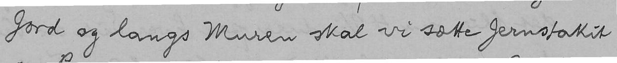Jord og langs Muren skal vi sætte Jernstakit
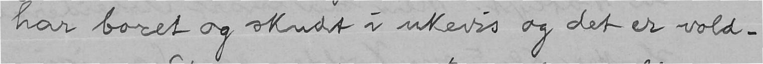har boret og skudt i ukevis og det er vold-
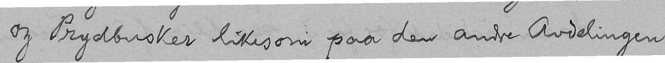og Prydbusker likesom paa den andre Avdelingen
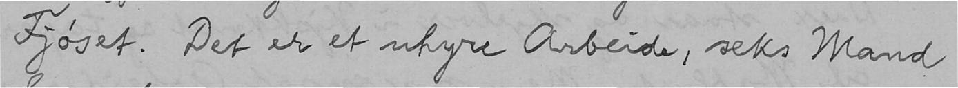Fjøset. Det er et uhyre Arbeide, seks Mand
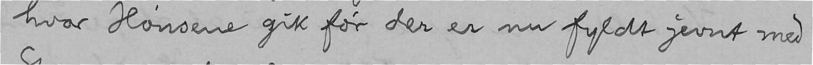hvor Hønsene gik før der er nu fyldt jevnt med
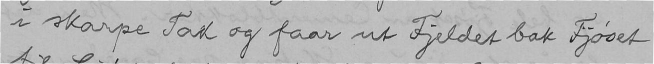i skarpe Tak og faar ut Fjeldet bak Fjøset
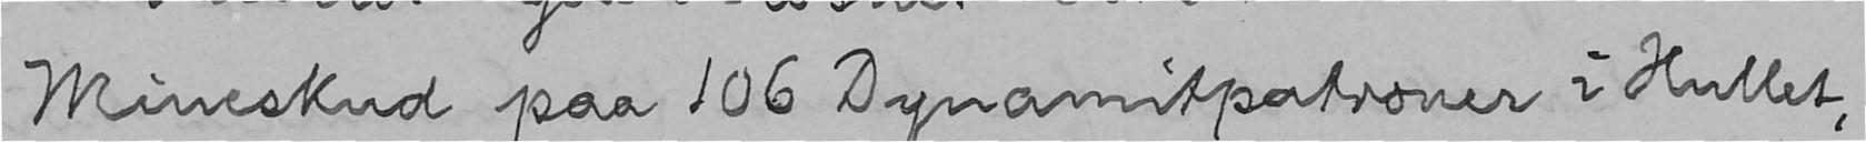Mineskud paa 106 Dynamitpatroner i Hullet,
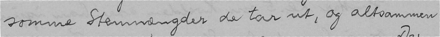somme stenmængder de tar ut, og altsammen
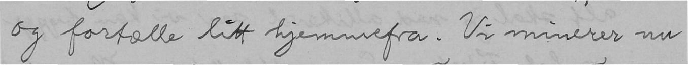og fortælle litt hjemmefra. Vi minerer nu
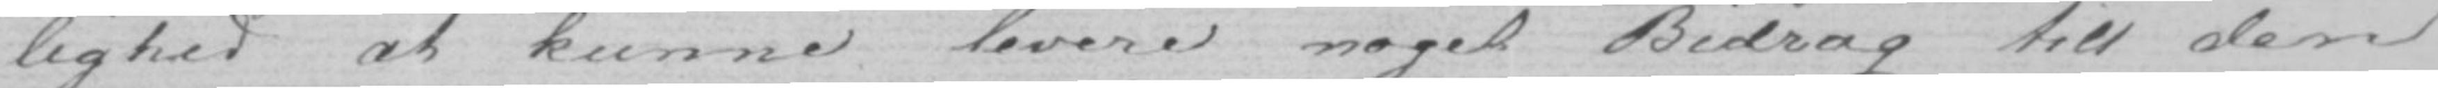lighed at kunne levere noget Bidrag till den
